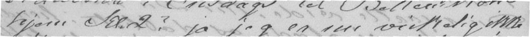hjem Kl.2? ja jeg er nu virkelig ikke
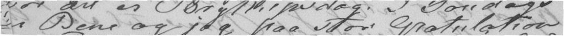var Bene og jeg paa stor Gratulation
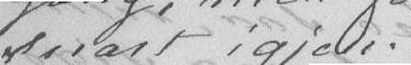snart igjen.
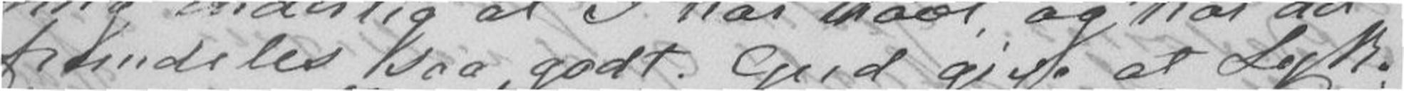fremdeles saa godt. Gud give at Lyk-
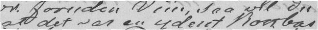at det var en yderst kostbar
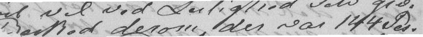Besked derom der var 144 Per-
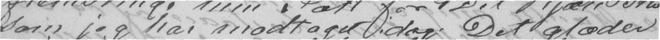som jeg har modtaget idag. Det glæder
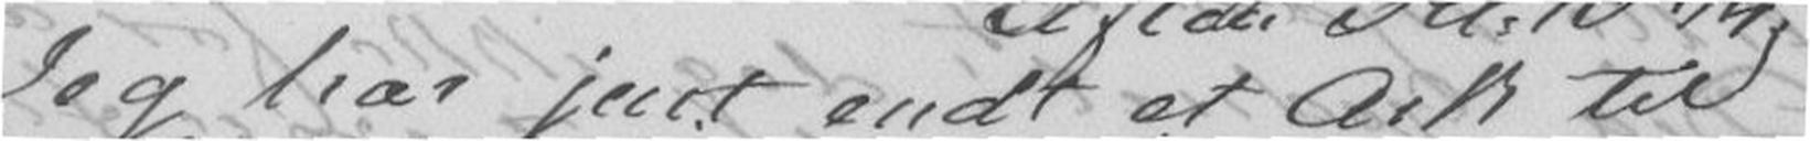Jeg har just endt et Ark til
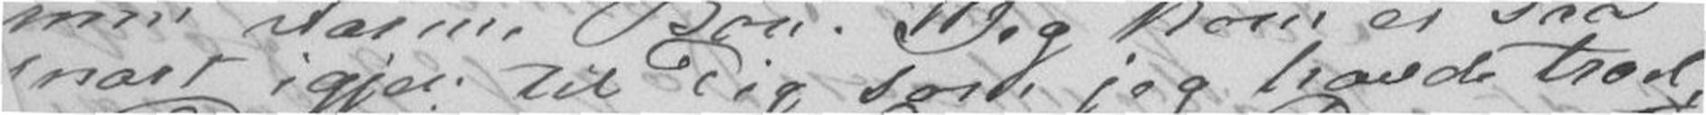snart igjen til Dig som jeg havde troet,
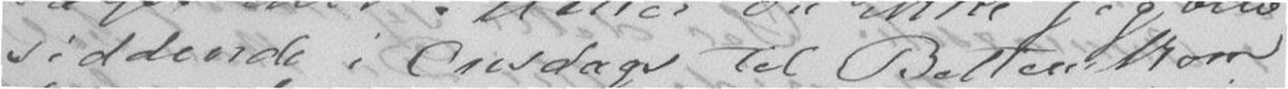siddende i Onsdags til Betten kom
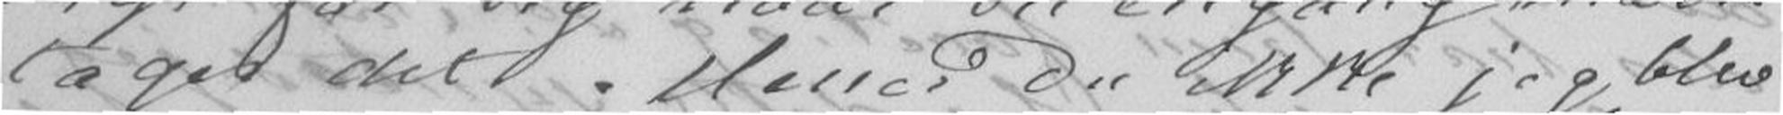tager det. Mener Du ikke jeg blev
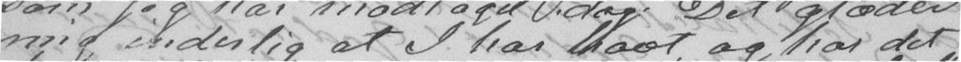mig inderlig at I har havt, og har det
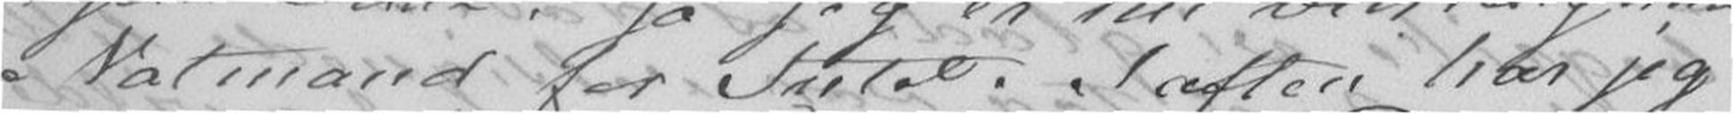Natmand for Intet. I aften har jeg
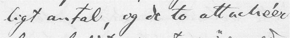ligt antal, og de to attachéer
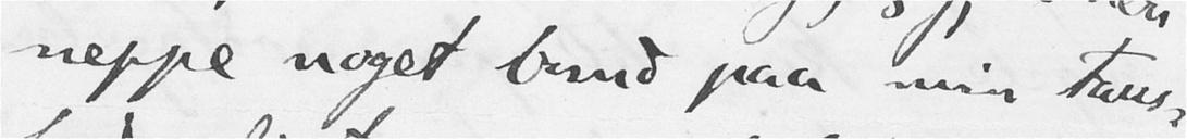neppe noget brud paa min taus-
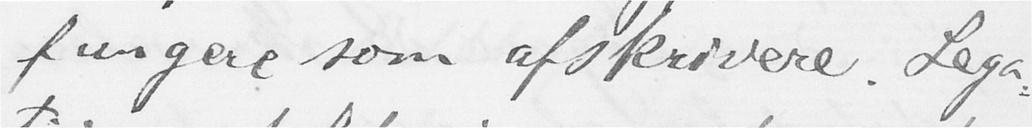fungere som afskrivere. Lega-
